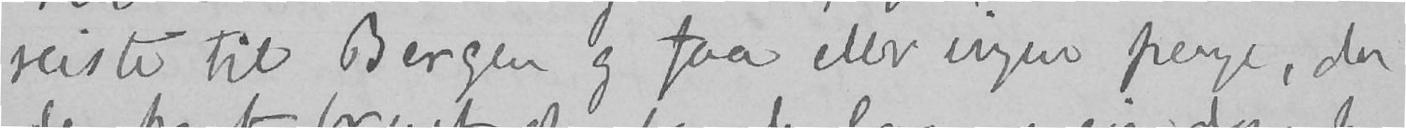reiste til Bergen og faa eller ingen penge, da
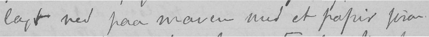lagt ned paa maven med et papir foran
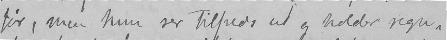før, men hun ser tilfreds ud og holder regn-
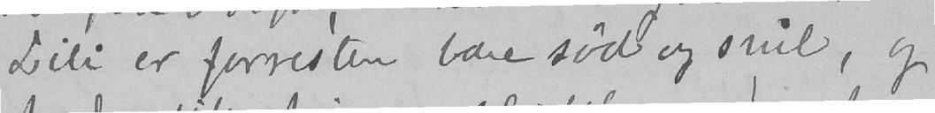Lili er forresten bare sød og snil, og
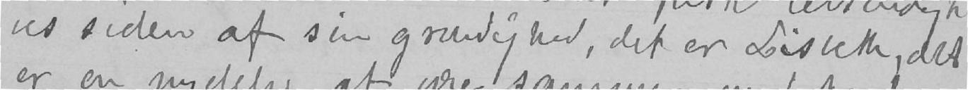ved siden af sin grundighed, det er Lisbeth, det
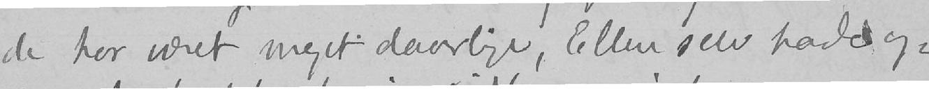de har været meget daarlige, Ellen selv havde og-
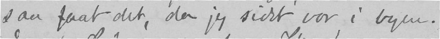saa faat det, da jeg sidst var i byen.
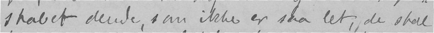skabet derude, som ikke er saa let, de skal
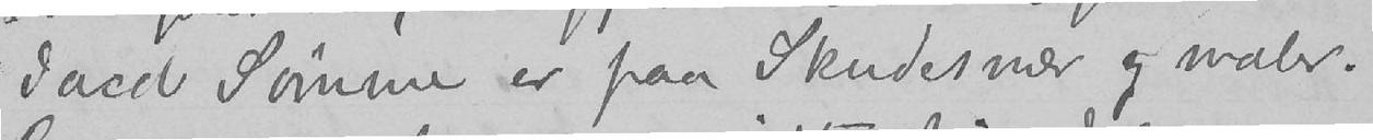Jacob Sømme er paa Skudesnæs og maler.
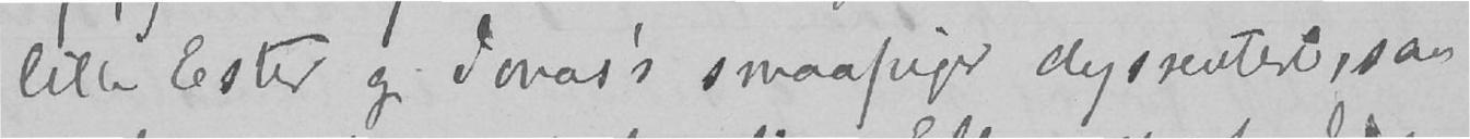lille Ester og Jonas's smaapiger dyssenteri saa
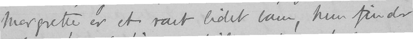Margrethe er et rart lidet barn, hun finder
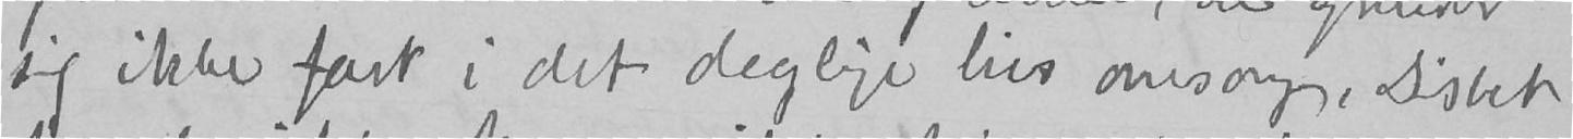sig ikke fast i det daglige livs omsorg, Lisbeth
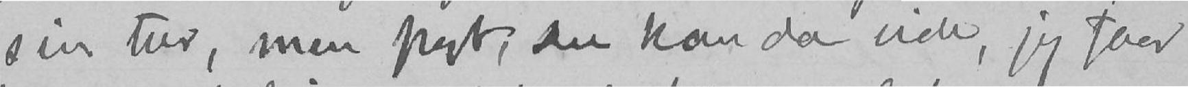sin tur, men pyt, du kan da vide, jeg faar
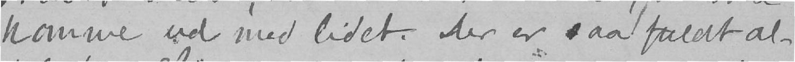komme ud med lidet. Der er saa fuldt al
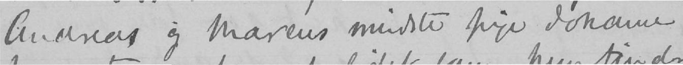Andreas og Marens mindste pige Johanne
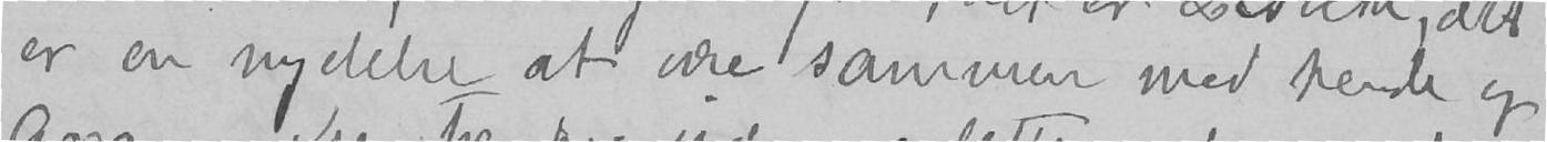er en nydelse at være sammen med hende og
